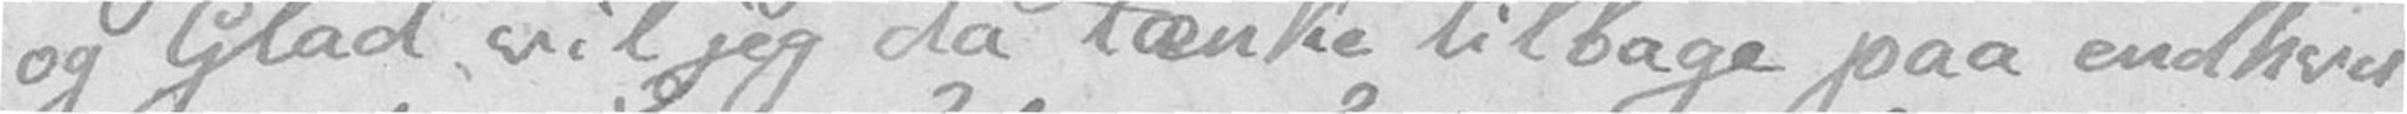og Glad vil jeg da tænke tilbage paa endhver
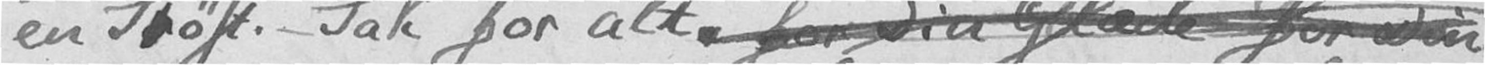en Trøst. – Tak for alt. for din Glæde for din
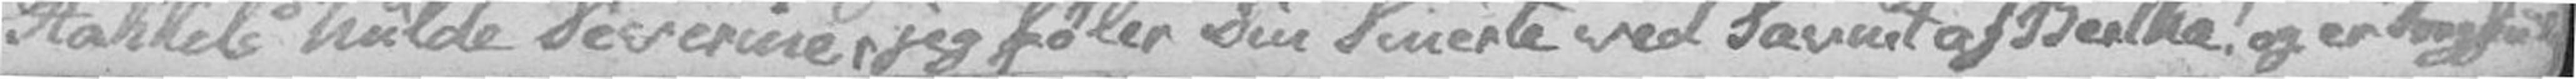Stakkels hulde Severine, jeg føler Din Smerte ved Savnet af Bertha! og er Sorgfuld
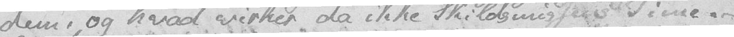dem, og hvad virker da ikke Skildsmissens Time. –
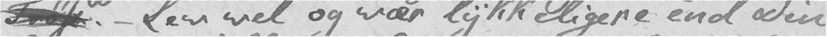Trøst. – Lev vel og vær lykkeligere end Din
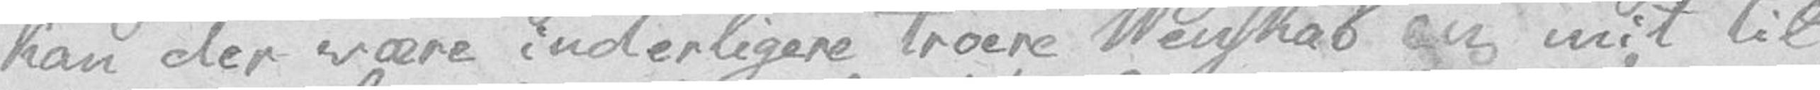kan der være inderligere troere Venskab en mit til
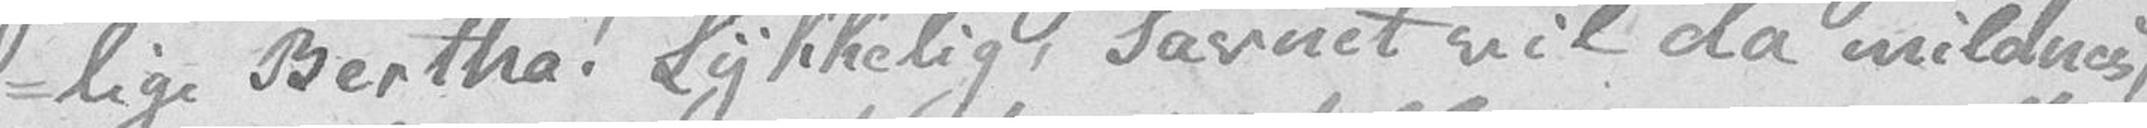-lige Bertha! Lykkelig, Savnet vil da mildnes,
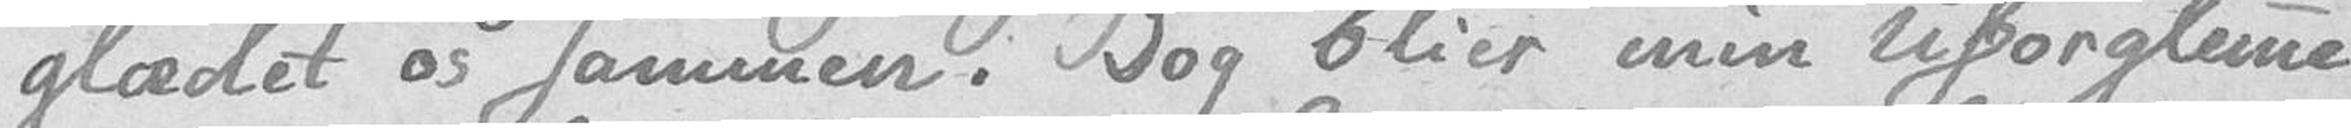glædet os sammen. Dog blier min uforglemme
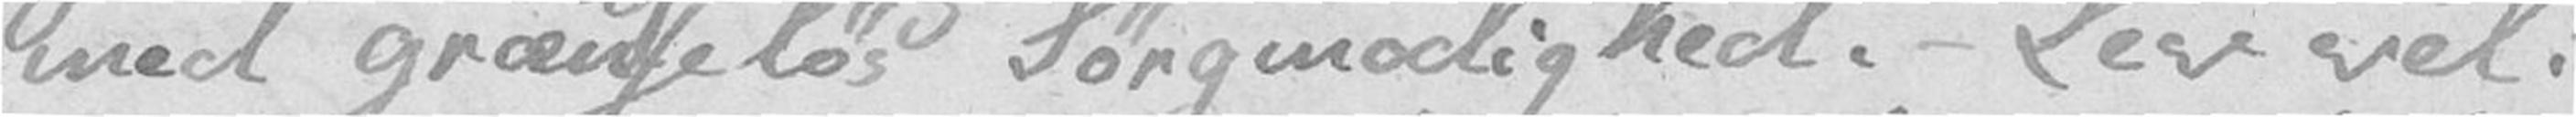med grænseløs Sørgmodighed. – Lev vel!
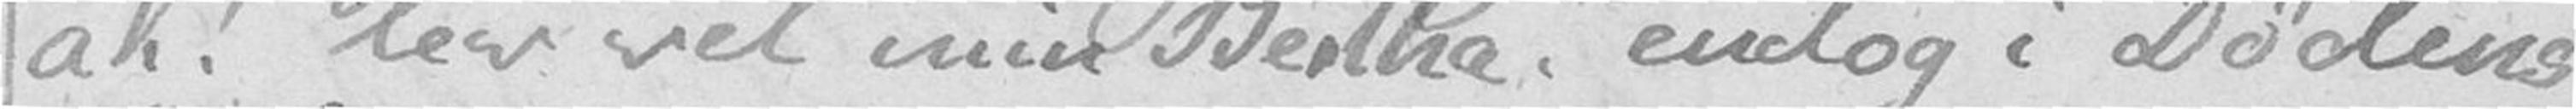ak! lev vel min Bertha! endog i Dødens
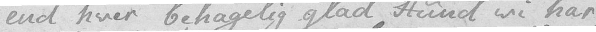end hver behagelig glad Stund vi har
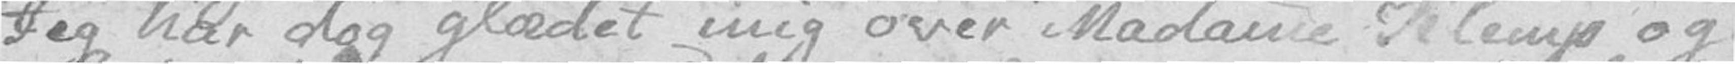Jeg har dog glædet mig over Madame Klemp og
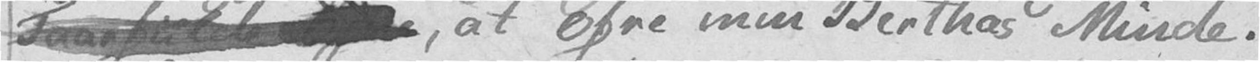Taarfulde Øjne, at ofre min Berthas Minde.
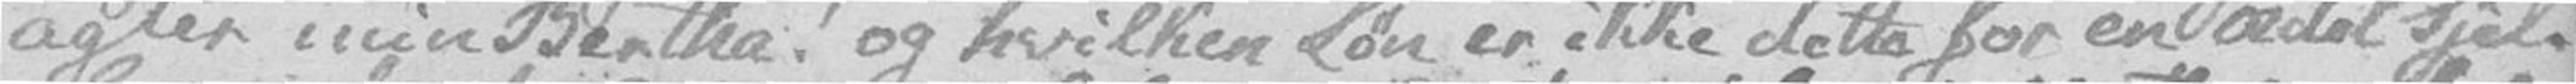agter min Bertha! og hvilken Løn er ikke dette for en ædel Sjel.
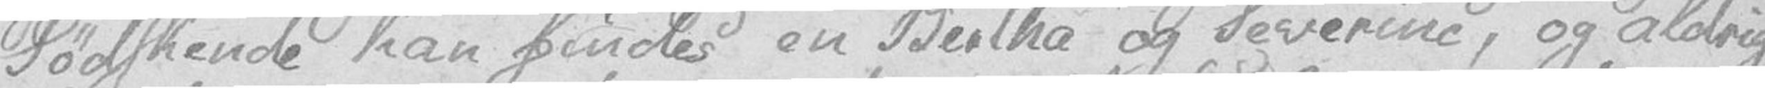Sødskende kan findes en Bertha og Severine, og aldrig
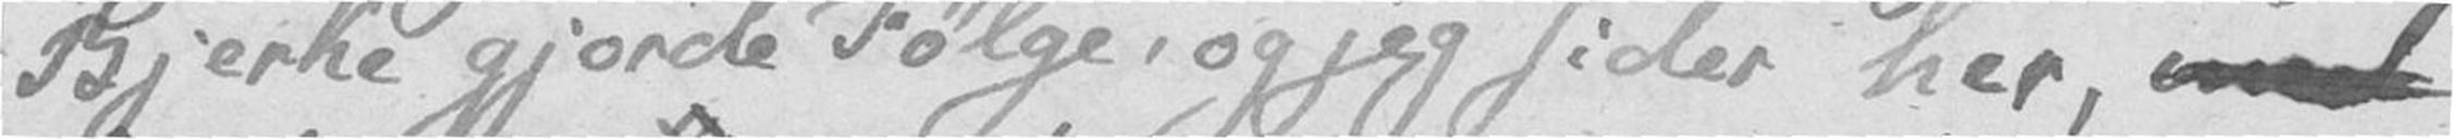Bjerke gjorde Følge, og jeg sider her, med
